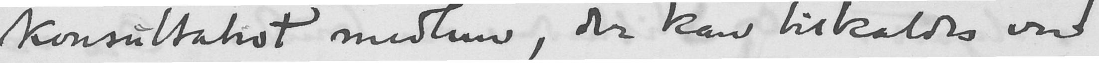konsultativt medlem, der kan tilkaldes ved
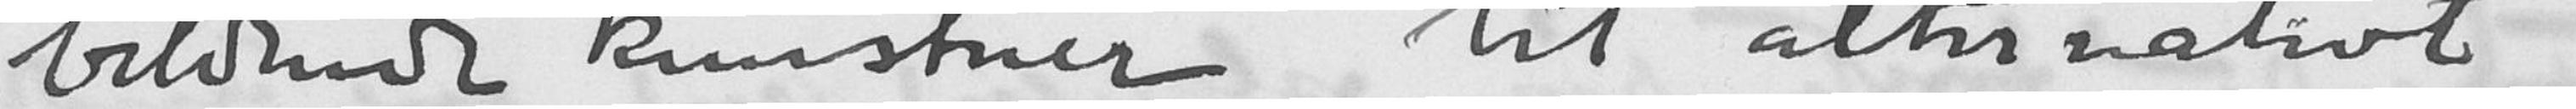"bildende kunstner" til alternativt
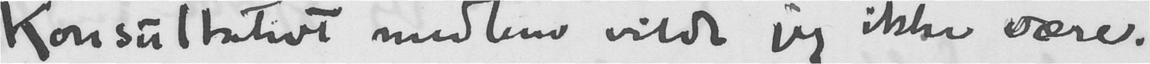Konsultativt medlem vilde jeg ikke være.
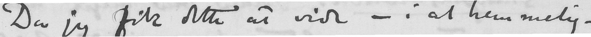Da jeg fik dette at vide - i al hemmelig-
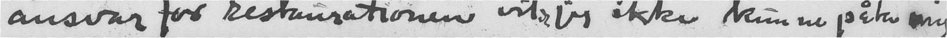ansvar for restaurationen vil jeg ikke kunne påta mig
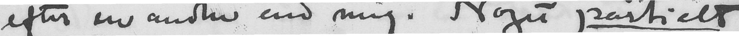efter en anden end mig. Noget partielt
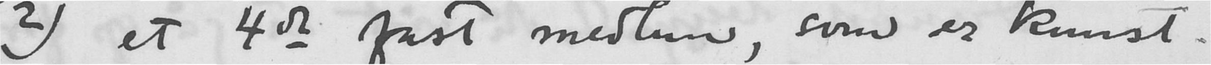2) et 4de fast medlem, som er kunst-
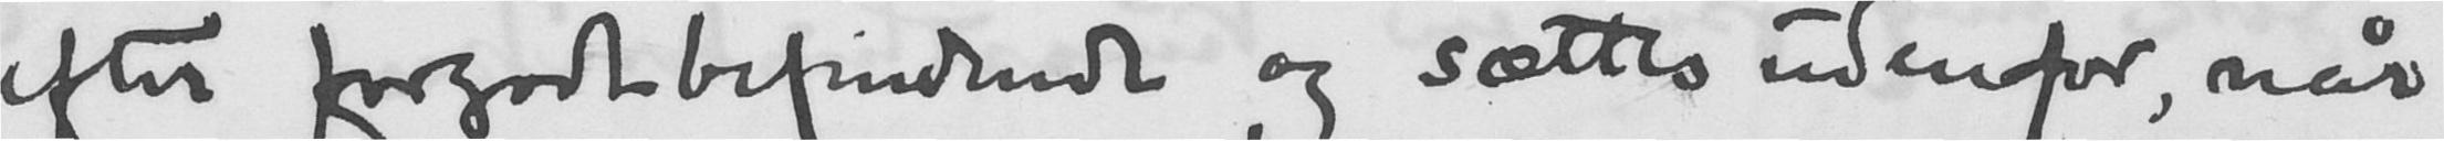efter forgodtbefindende og sættes udenfor, når
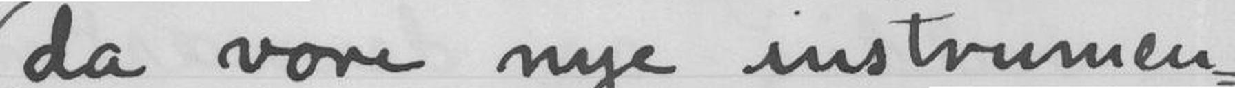da vore nye instrumen-
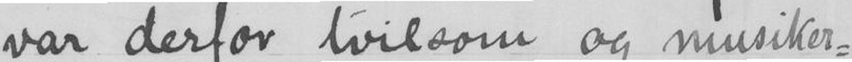var derfor tvilsom og musiker-
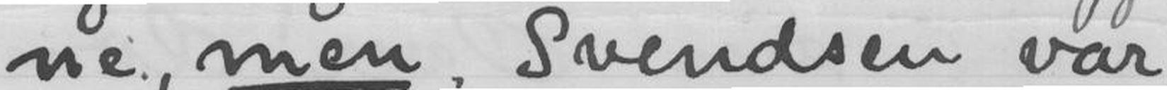ne, men, Svendsen var
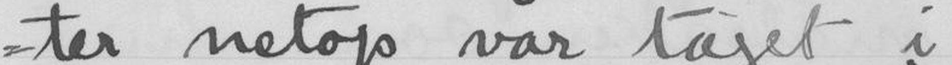ter netop var taget i
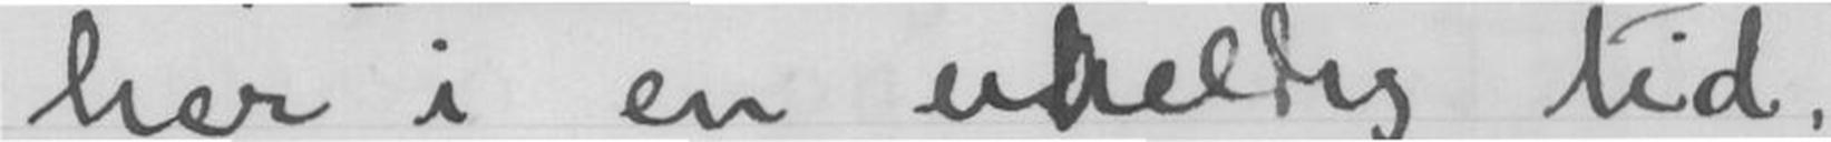her i en uheldig tid,
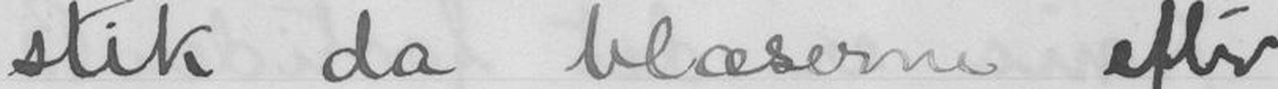stik da blæserne efter
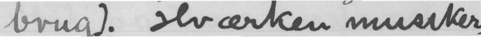brug). Hværken musiker
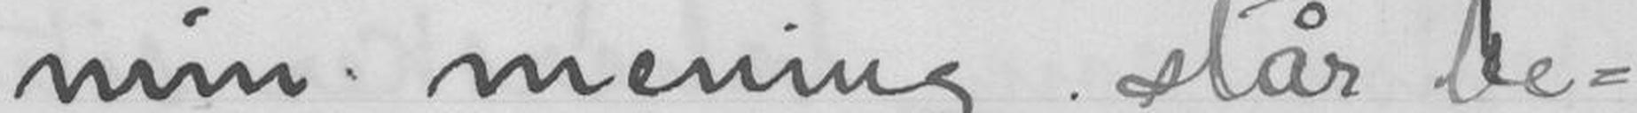min mening står be-
